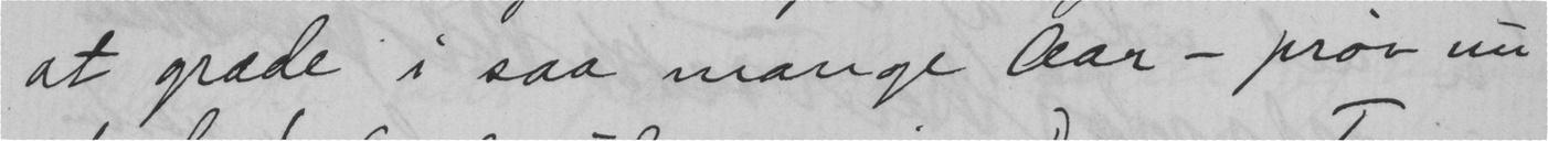at græde i saa mange aar - prøv nu
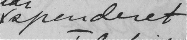spenderet
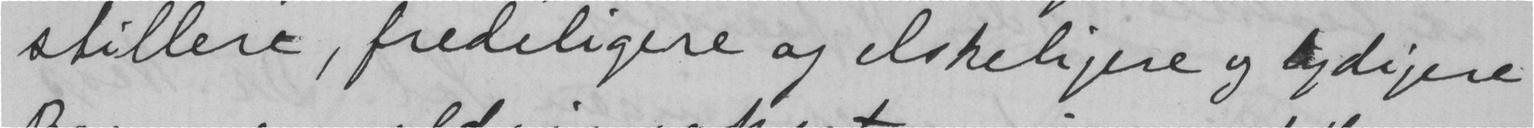stillere, fredeligere og elskeligere og lydigere
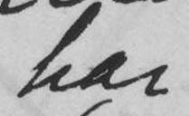har
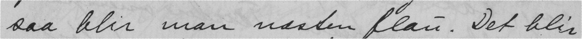saa blir man næsten flau. Det blir
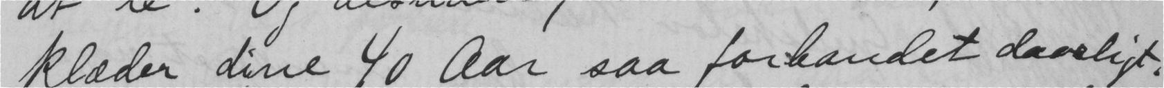klæder dine 40 Aar saa forbandet daarligt.
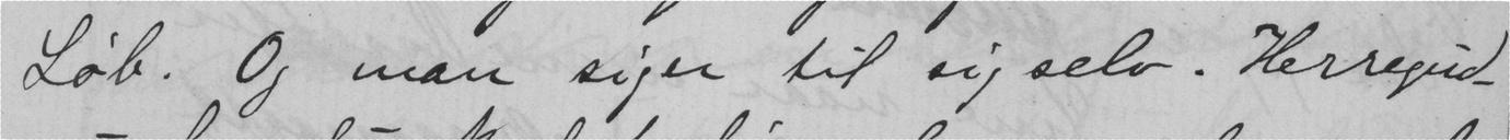Løb. Og man siger til sig selv. Herregud -
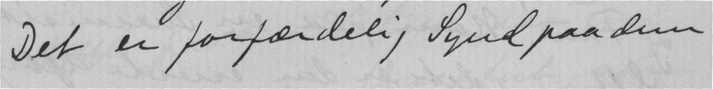Det er forfærdelig Synd paa dem
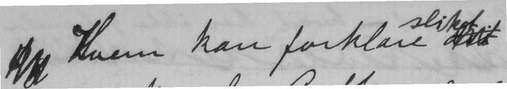Hvem kan forklare
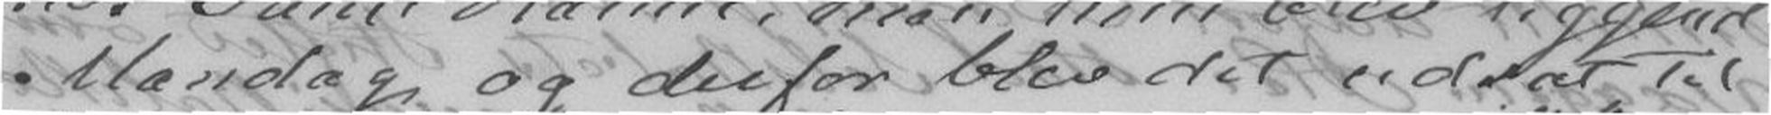Mandag og derfor blev det udsat til
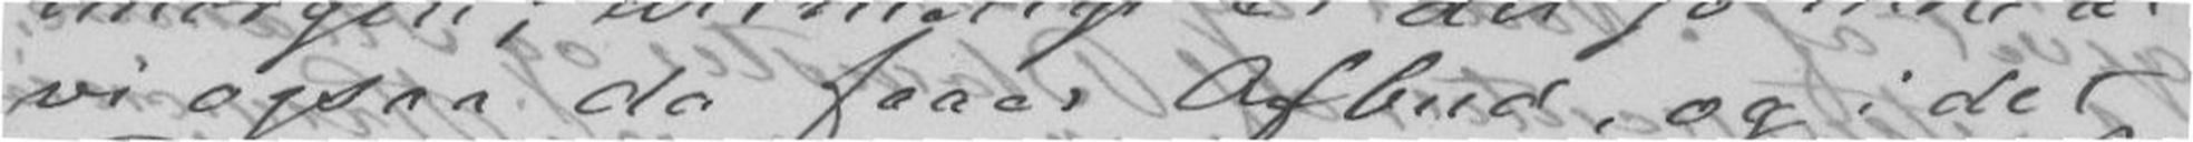vi ogsaa da faaer Afbud, og i det
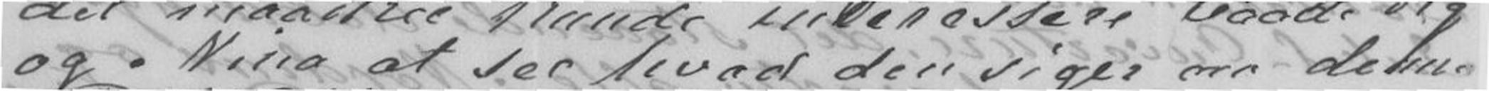og Nina at see hvad den siger omnu denne
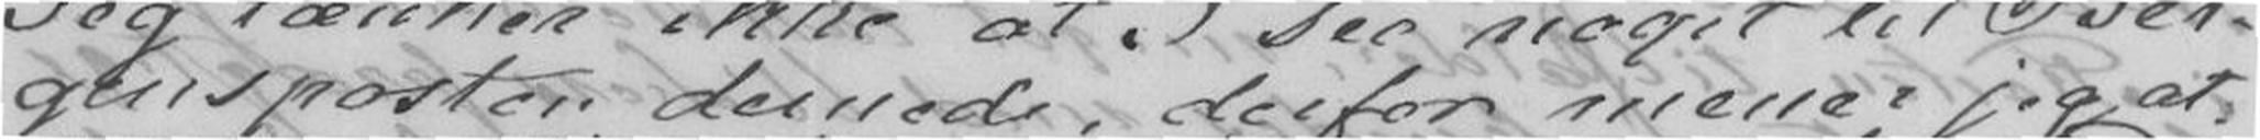gensposten dernede, derfor mener jeg at
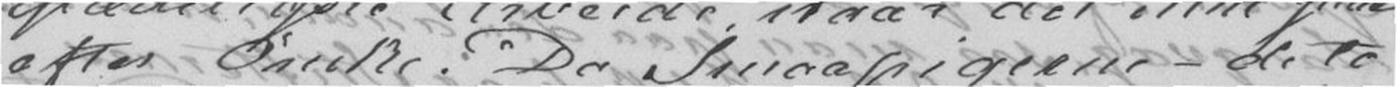efter Ønske. Da Smaapigerne - de to
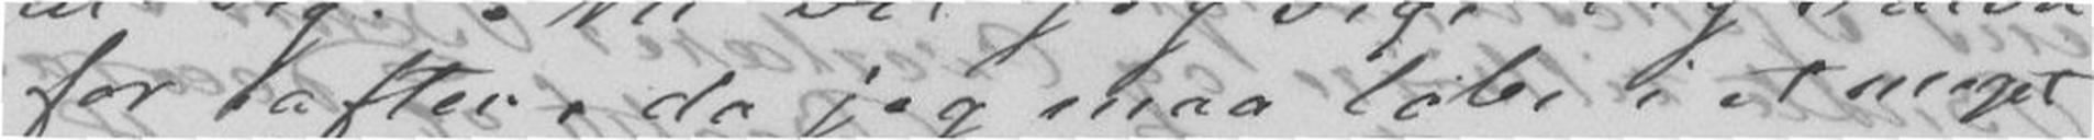for iaften, da jeg maa løbe i et meget
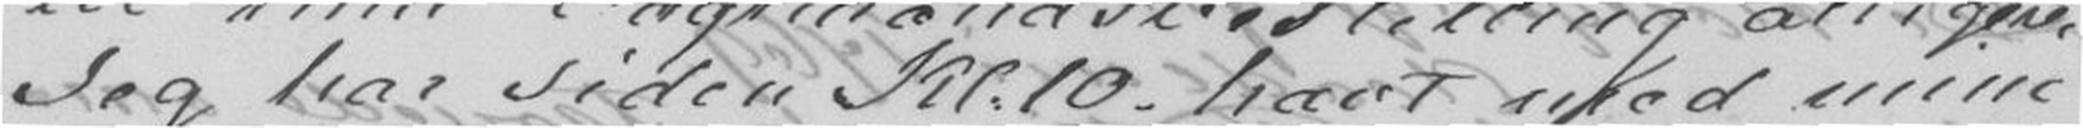Jeg har siden Kl.10. havt med mine
